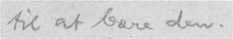til at bære den.
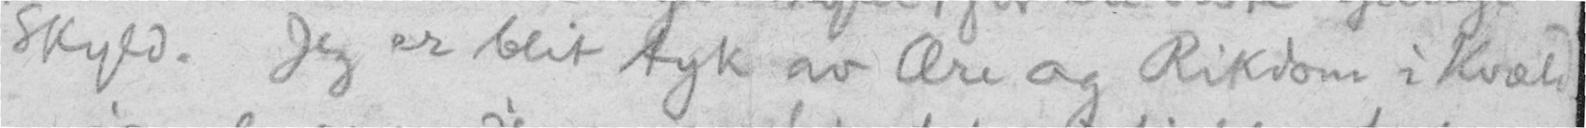Skyld. Jeg er blit tyk av Ære og Rikdom i Kvæld
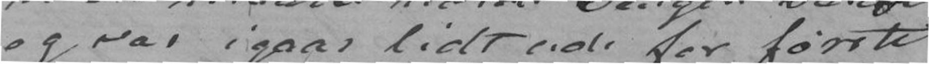og var igaar lidt ude for første
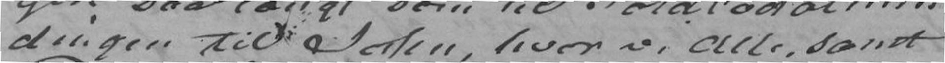dingen til John, hvor vi Alle samt
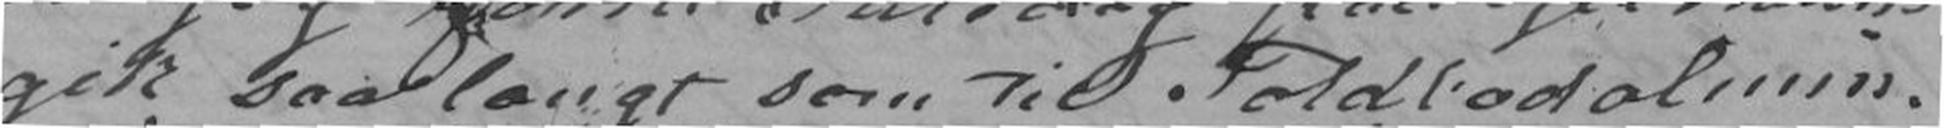gik saalangt som til Toldbosalmen-
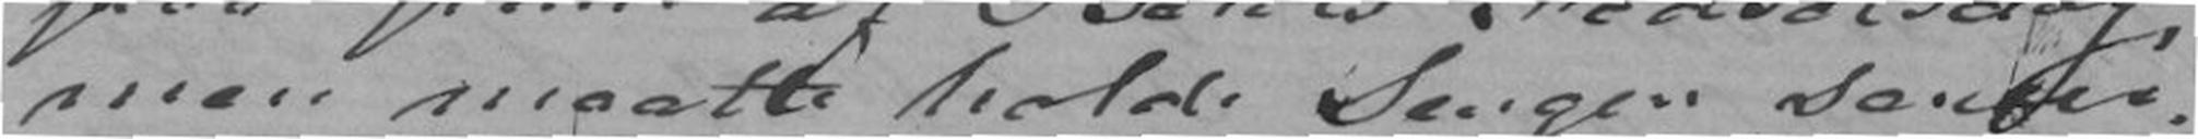men maatte holde Sengen senere,
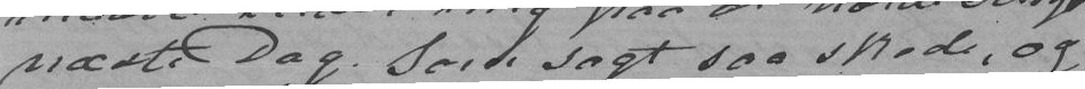næste Dag. Som sagt saa skede, og
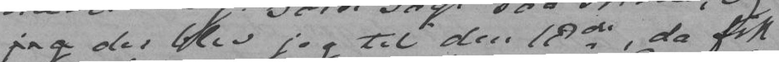jeg der blev jeg til den 18de, da fik
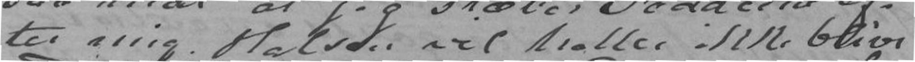ter mig. Halsen vil heller ikke blive
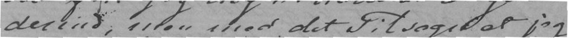derind, men med det Tilsagn at jeg
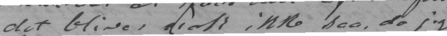det bliver nok ikke saa, da jeg
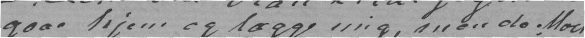gaae hjem og lægge mig, men da Moer
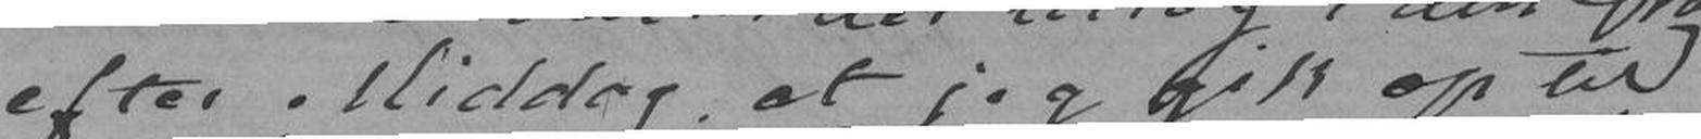efter Middag, at jeg gik op til
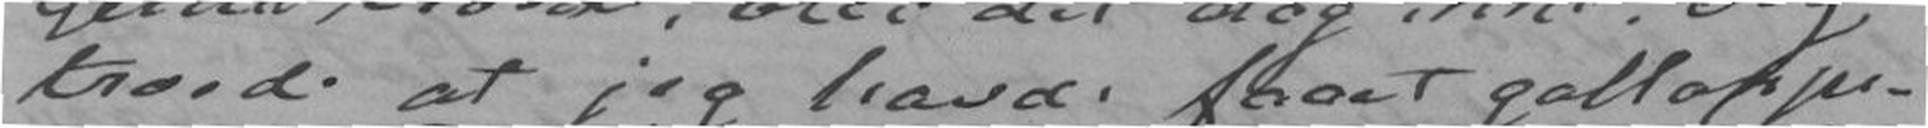troed at jeg havde faaet galloppe-
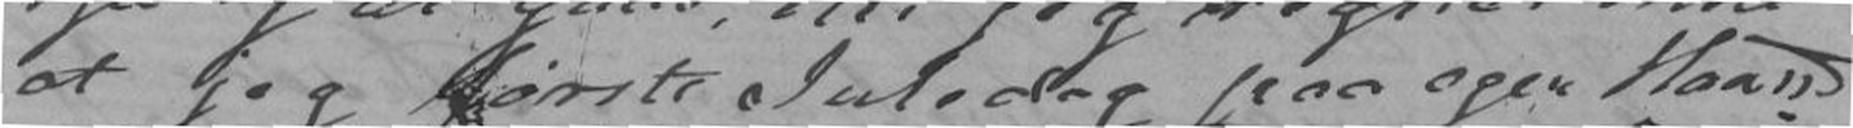at jeg første Juledag paa egen Haand
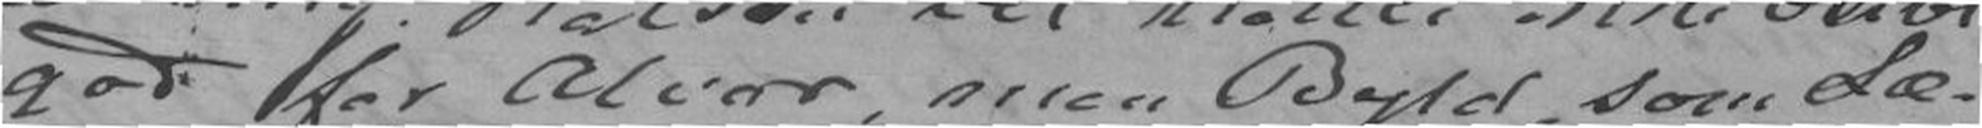god for Alvor, men Byld, som Læ-
